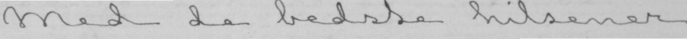Med de bedste hilsener
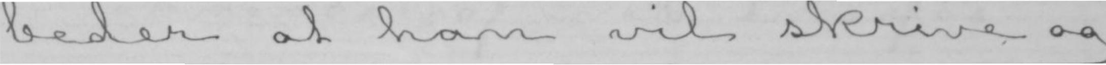beder at han vil skrive og
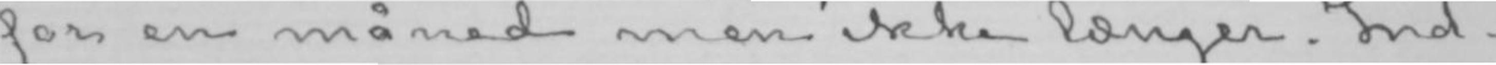for en måned men ikke længer. Ind-
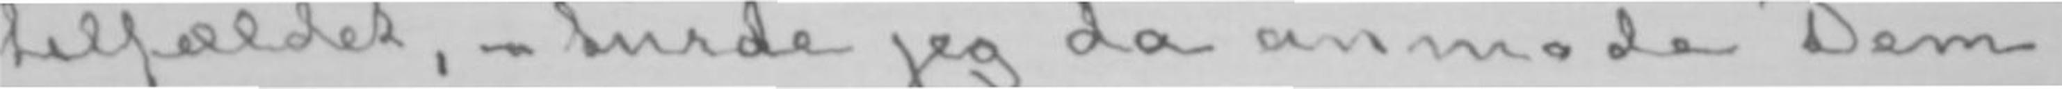tilfældet,- turde jeg da anmode Dem
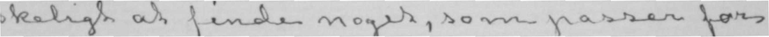skeligt at finde noget, som passer for
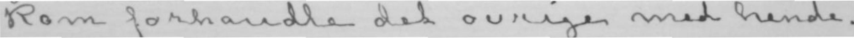Rom forhandle det øvrige med hende.
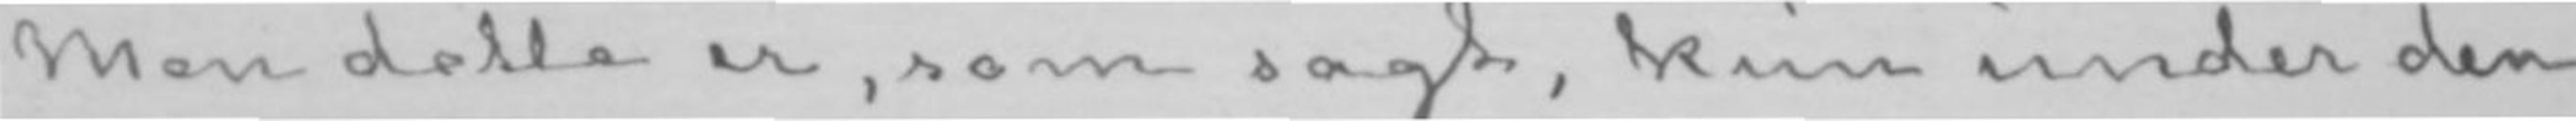Men dette er, som sagt, kun under den
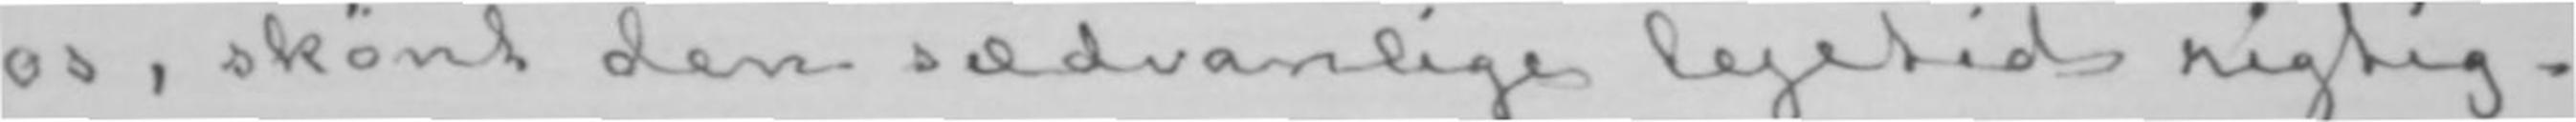os, skønt den sædvanlige lejetid rigtig-
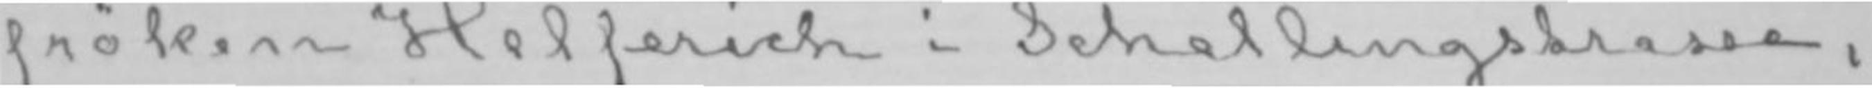frøken Helferich i Schellingstrasse,
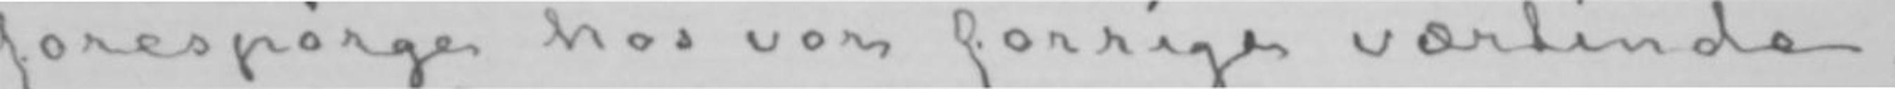forespørge hos vor forrige værtinde,
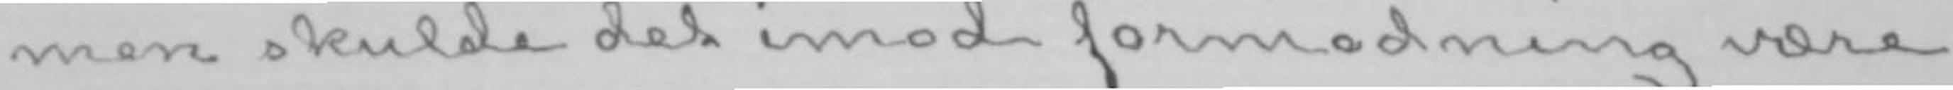men skulde det imod formodning være
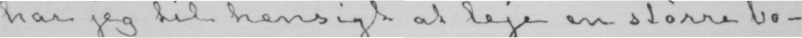har jeg til hensigt at leje en større bo-
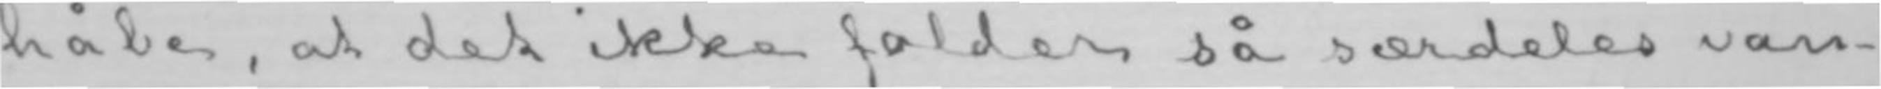håbe, at det ikke falder så særdeles van-
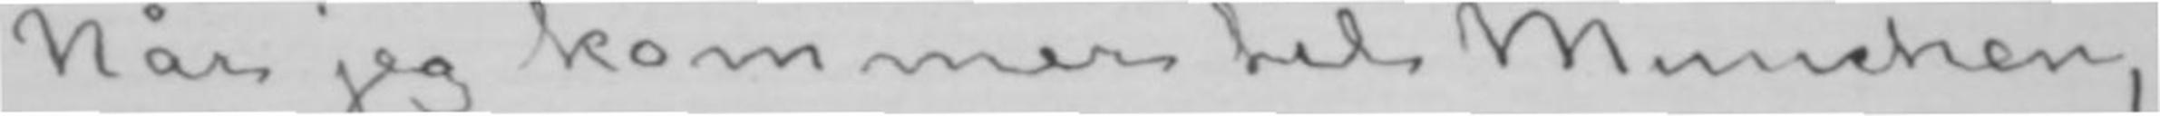Når jeg kommer til München,
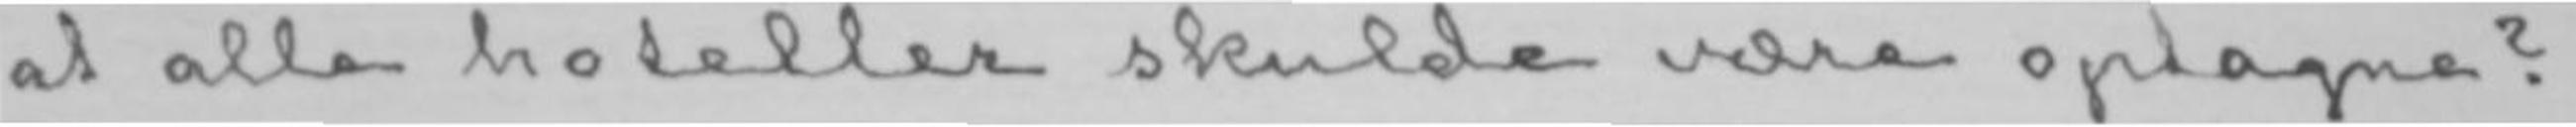at alle hoteller skulde være optagne?
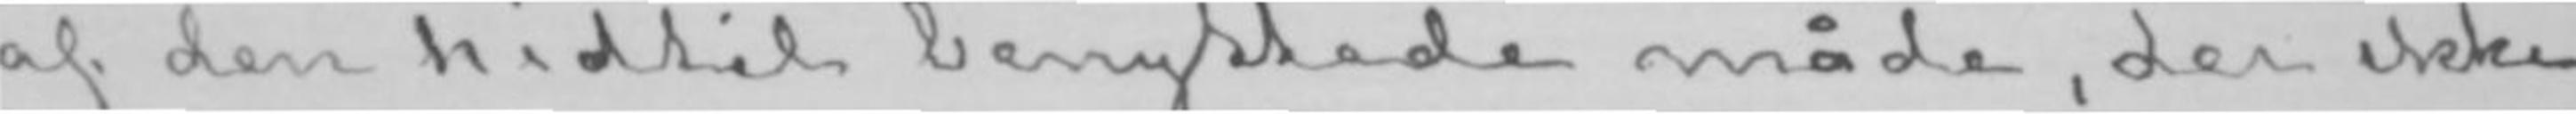af den hidtil benyttede måde, der ikke
